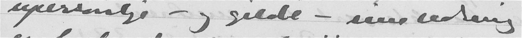upersonlige - og gilde - redning
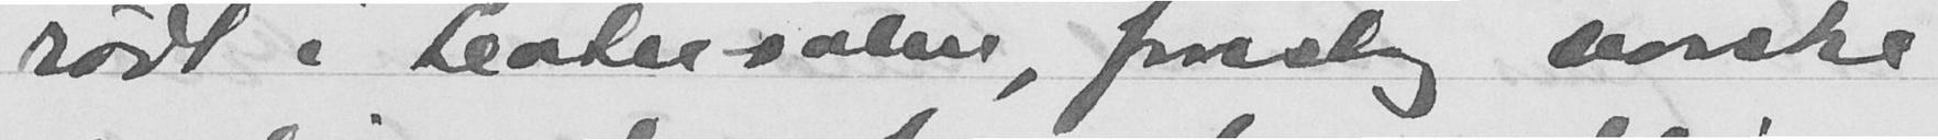rødt i teatersalen, foreslog norske
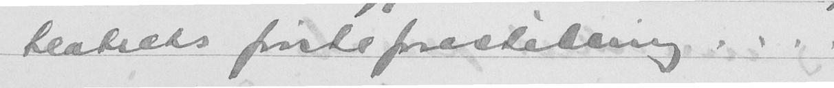teatrets første forestilning....
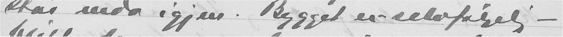står enda igjen. Bygget er selvfølgelig -
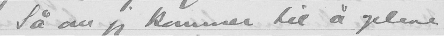Så om jeg kommer til å opleve
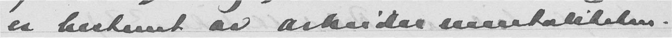er bestemt av arbeidermentaliteten.
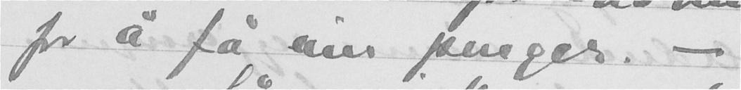for å få inn penger. -
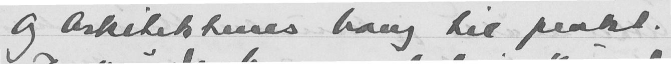Og Arkitektenes hang til prakt.
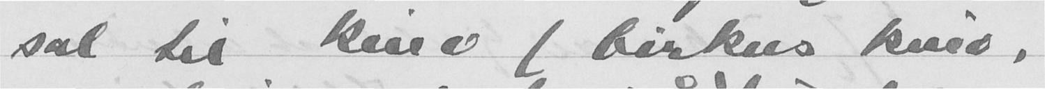sal til kino (birkus kino,
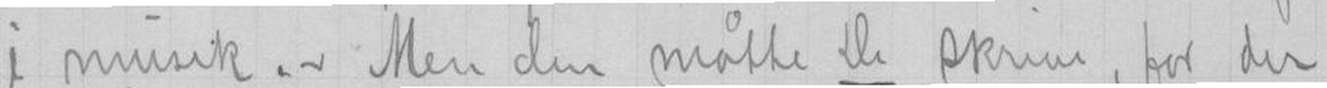i musik.- Men den måtte De skrive, for der
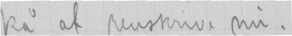på at renskrive nu.
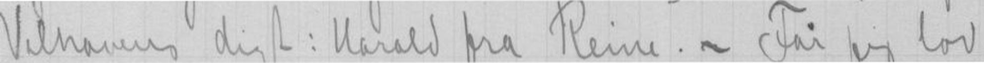Velhavens digt: Harald fra Reine.- Får jeg lov
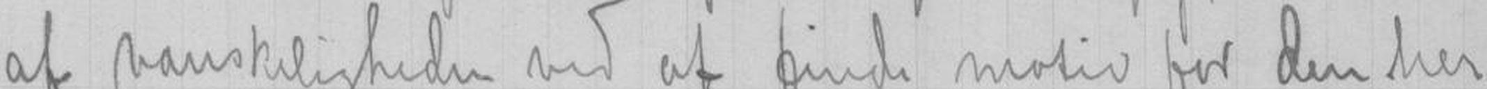af vanskeligheder ved af finde motiv for den her
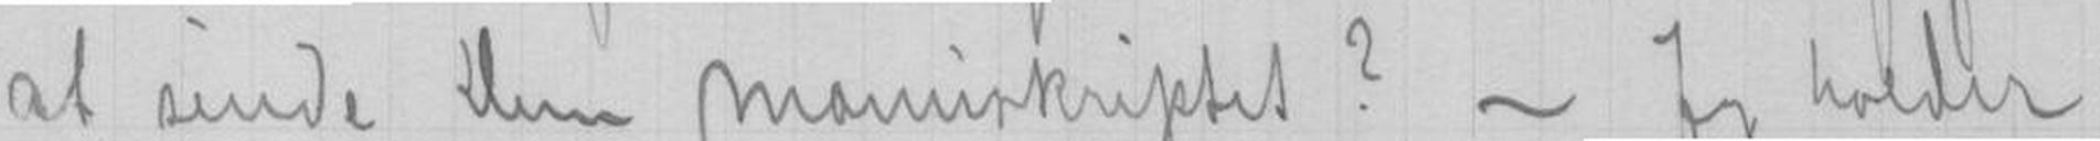at sende Dem manuskriptet ? - Jeg holder
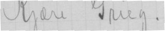Kjære Grieg.
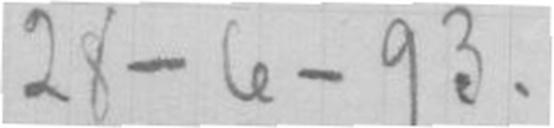28-6-93.
